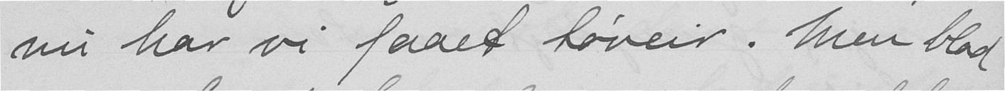nu har vi faaet tøveir. Men blod
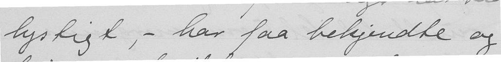lystigt ,- har faa bekjendte og
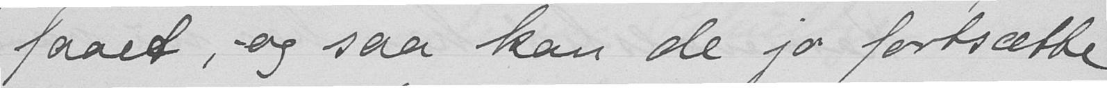faaet, og saa kan de jo fortsætte
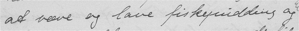at væve og lave fiskepudding og
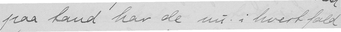paa tand har de nu i hvert fald
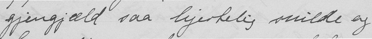gjengjæld saa hjertelig snilde og
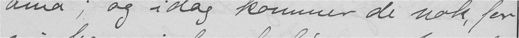ania; og i dag kommer de nok for
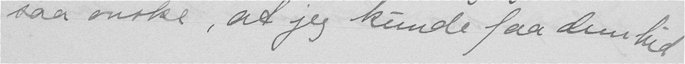saa ønske, at jeg kunde faa dem hid
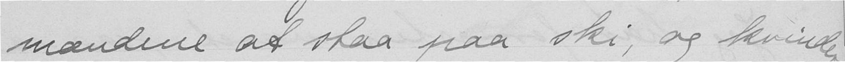mændene at staa paa ski, og kvindene
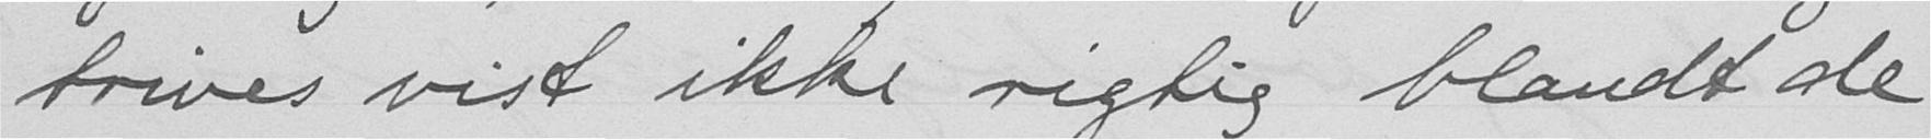trives vist ikke rigtig blandt de
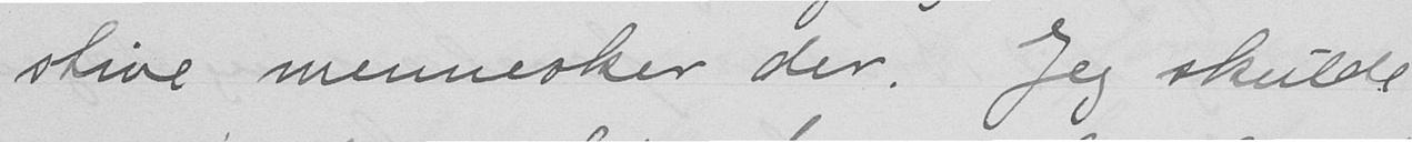stive mennesker der. Jeg skulde
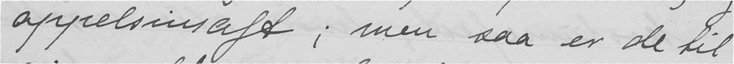appelsinsaft; men saa er de til
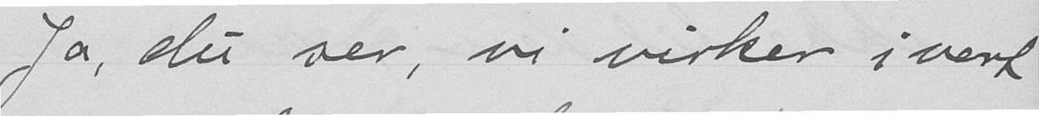Ja, du ser, vi virker i vert
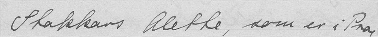Stakkars Alette, som er i Prag,
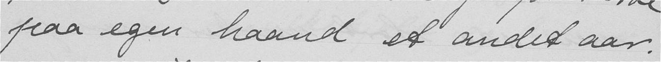paa egen haand et andet aar.
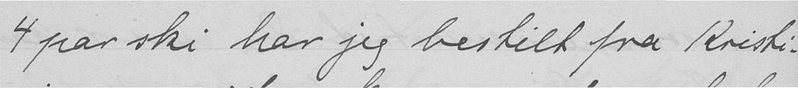4 par ski har jeg bestilt fra Kristi-
